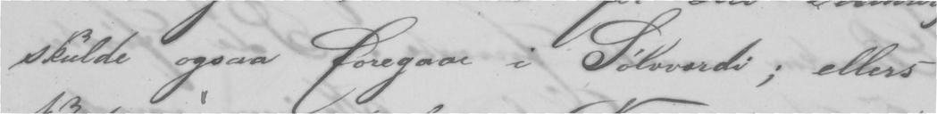skulde ogsaa foregaa i Sølvværdi; ellers
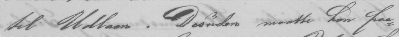til Udlaan. Desuden maatte han paa-
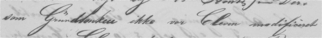som Grundtanken ikke var bleven modificeret
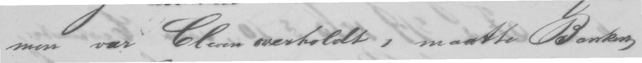men var bleven overholdt, maatte Banken
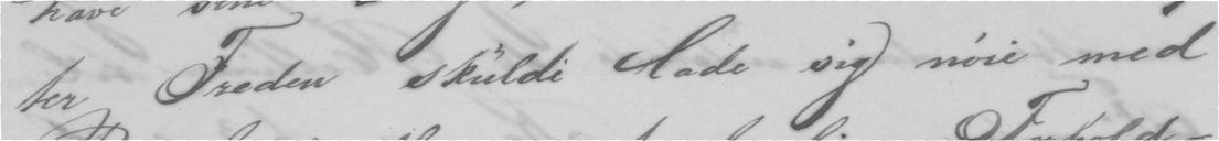ter Freden skulde lade sig nøie med
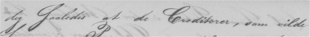dog saaledes at de Creditorer, som vilde
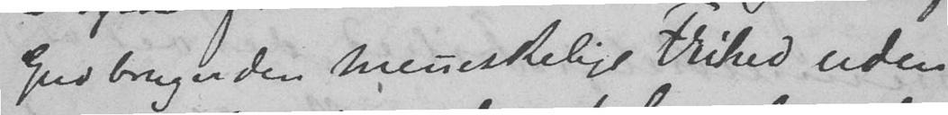Gud bruger den menneskelige Frihed uden
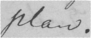plan.
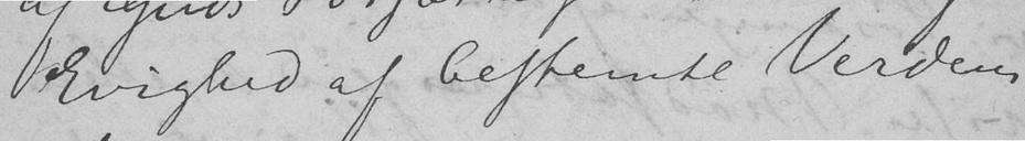Evighed af bestemte Verdens-
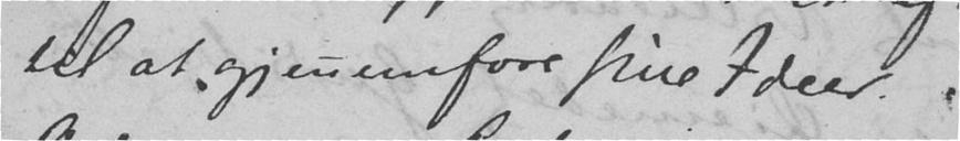til at gjennemføre sine Ideer.
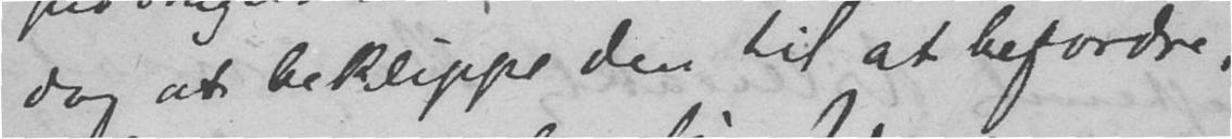dog at beklippe den til at befordre,
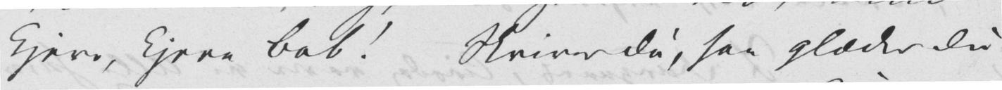kjere, kjere Bob! Skriver Du, saa glæder Du
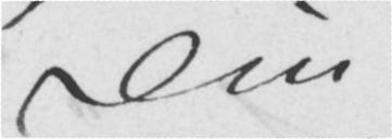Din
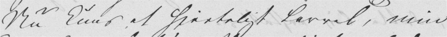Nu kuns et hjerteligt Levvel, min
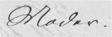Moder.
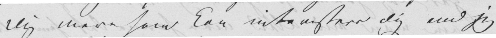Dig mere som kan interessere Dig end jeg.
